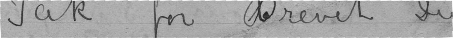Tak for Brevet Du
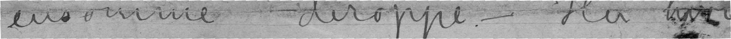ensomme deroppe – Hu hvor
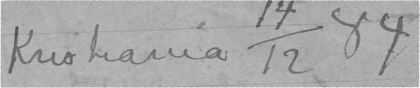Kristiania 14/12-84
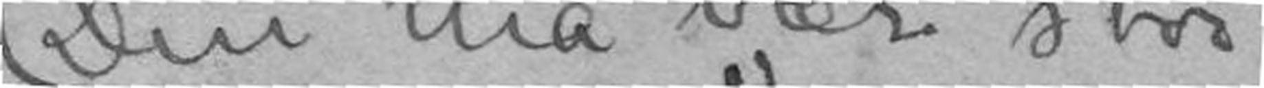(Den må være stor)
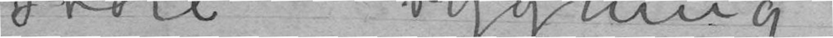store den bygning
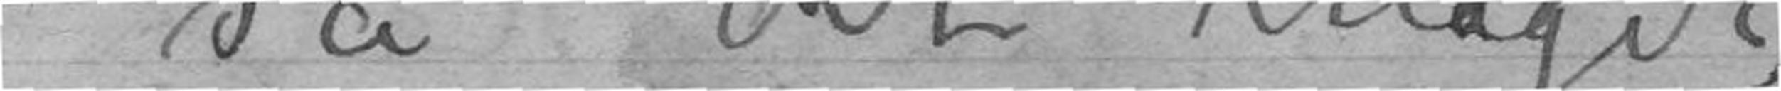så det knager.
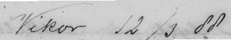Vikør 12/3 88
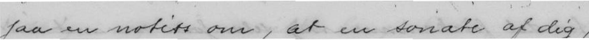saa en notis om, at en sonate af dig,
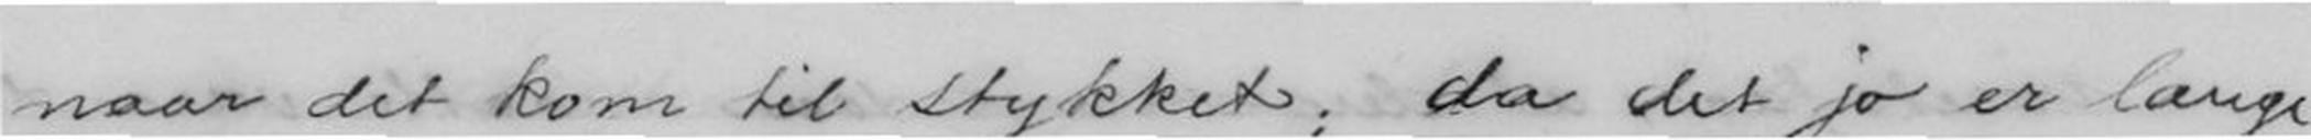naar det kom til stykket; da det jo er længe
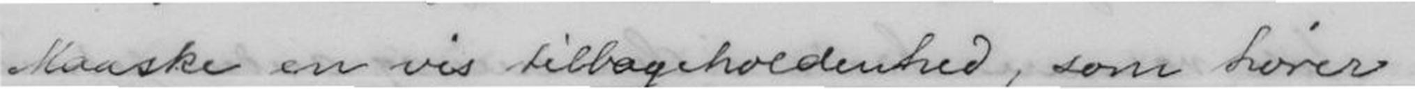Maaske en vis tilbageholdenhed, som hører
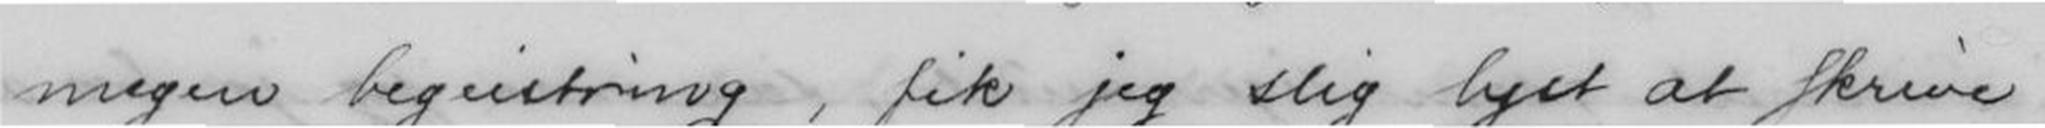megen begeistring, fik jeg slig lyst at skrive
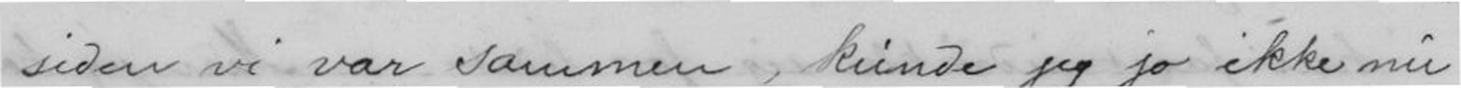siden vi var sammen, kunde jeg jo ikke nu
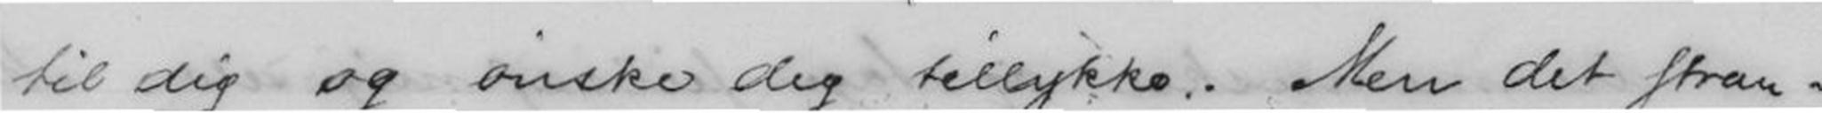til dig og ønske dig tillykke. Men det stran-
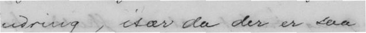udring, især da der er saa
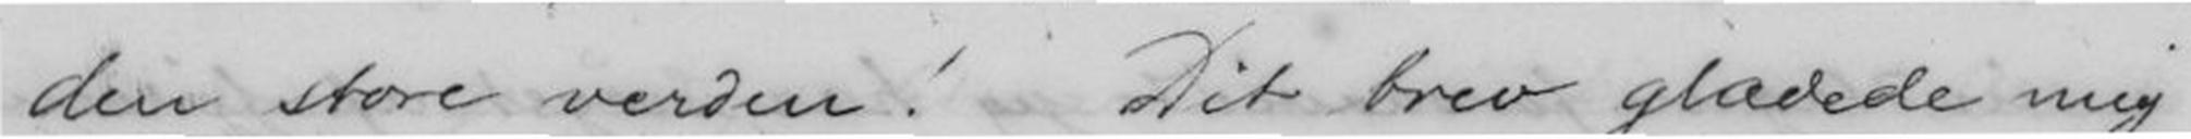den store verden! Dit brev glædede mig
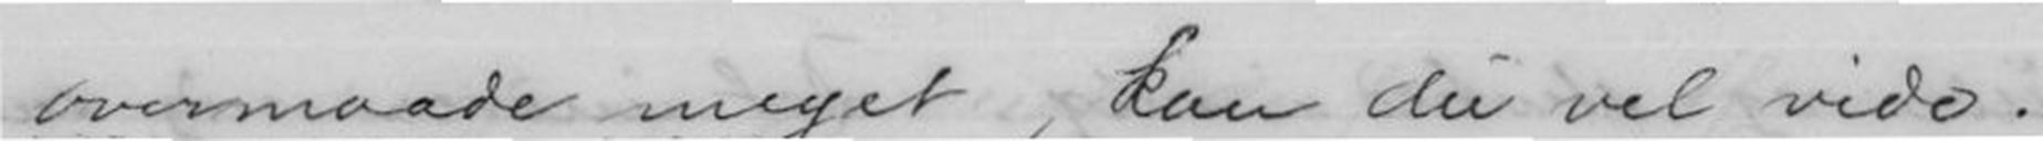overmaade meget, kan du vel vide, -
